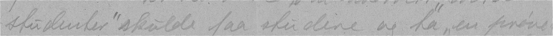studenter" skulde faa studere og ta "en prøve"
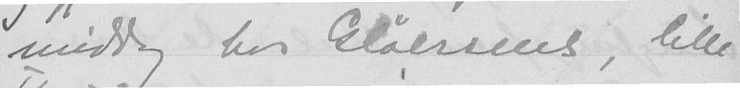middag hos Gløersens, lille
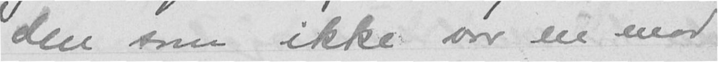den som ikke var en mod
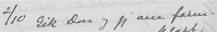2/10 Gik Don og jeg om form.
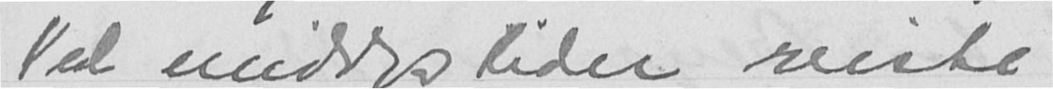Til middagstider reiste
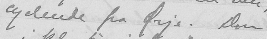cyclende fra Ørje. Don
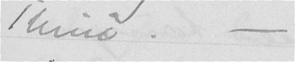Thrine. -
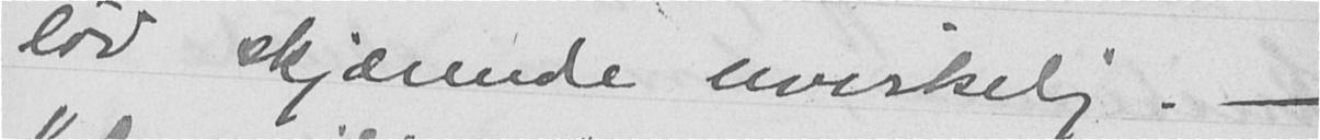lød skjærende uvirkelig. -
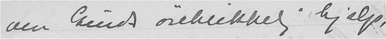om Guds øieblikkelig hjælp,
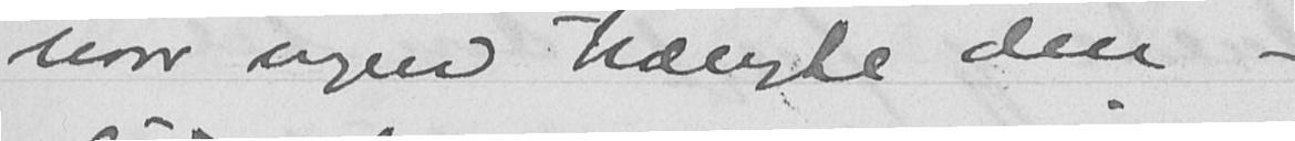naar nogen trængte den -
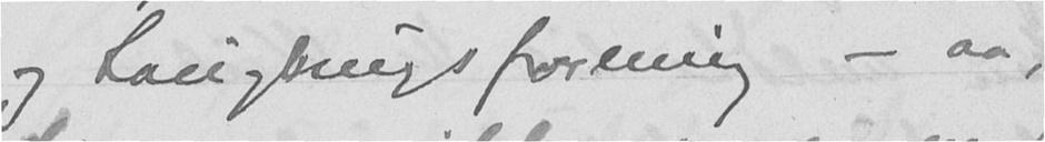og Saugbrugsforening - aa,
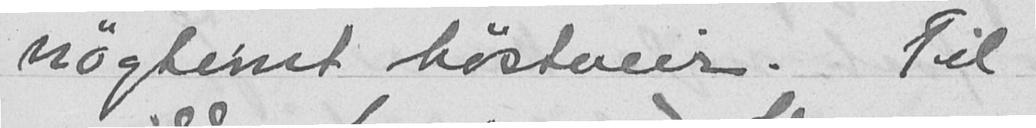nøgternt høstveir. Til
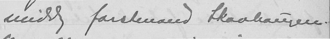middag forstmand Skovhaugen.
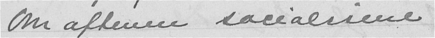Om aftenen socialisme
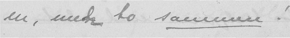en, men to sammen!
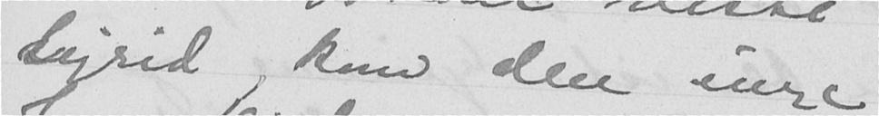Sigrid kom den unge
